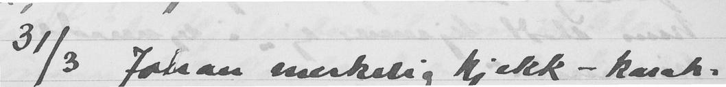31/3 Johan merkelig kjekk - karak-
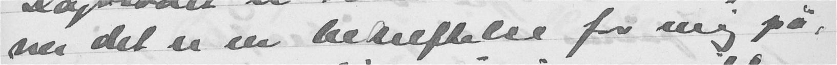ner det er en bekreftelse for mig på,
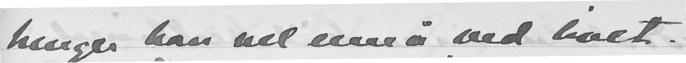henger han vel ennå ved livet.
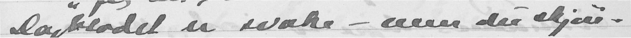Dagbladet er svake - men du skjøn-
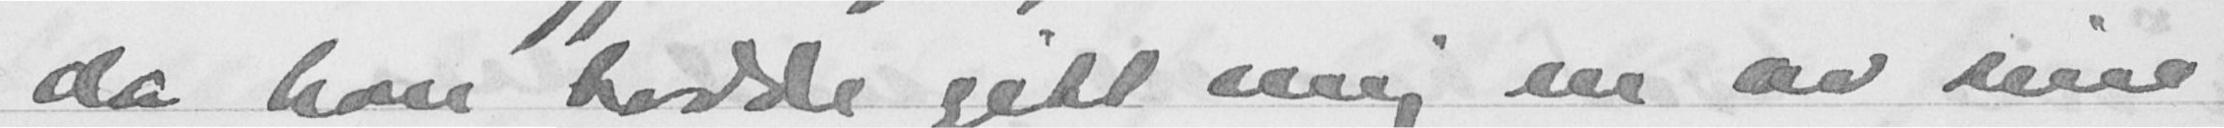da han hadde gitt mig en ev sine
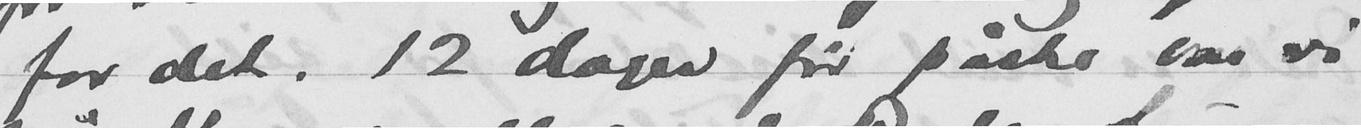for det. 12 dager før påske var vi
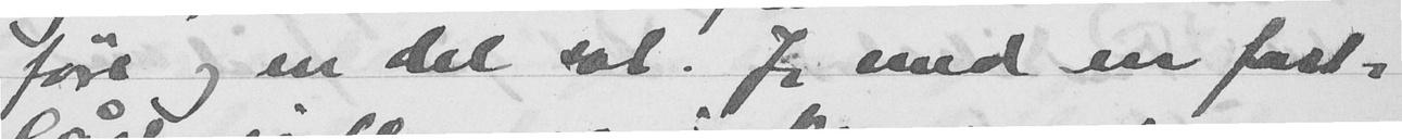føre og en del sol. Jeg med en fast-
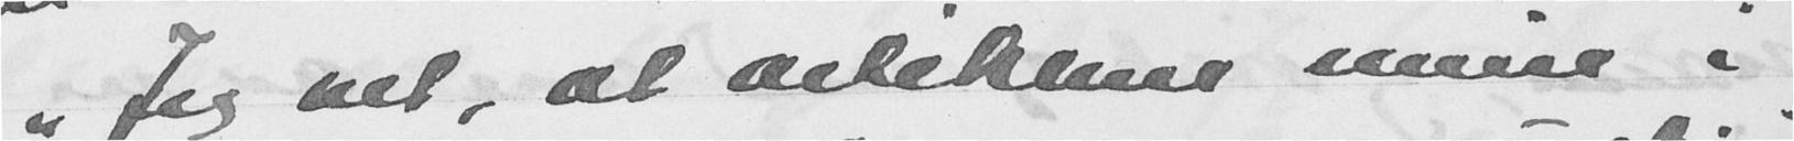"Jeg vet, at artiklene mine i
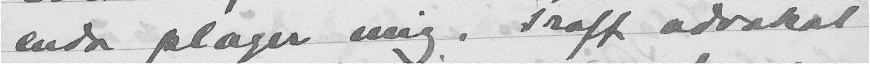enda plager mig. Traff advokat
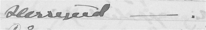Herregud - .
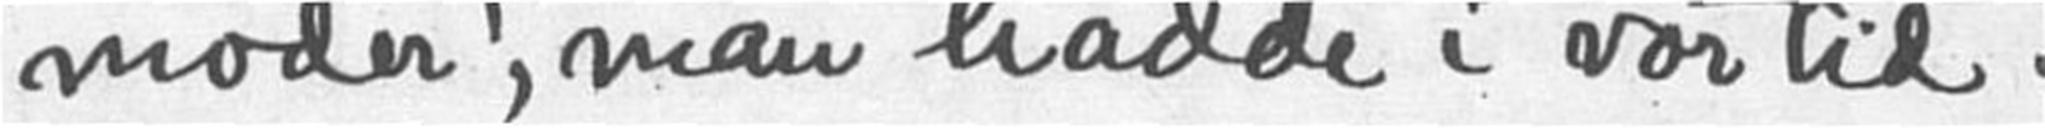moder, man hadde i vor tid.
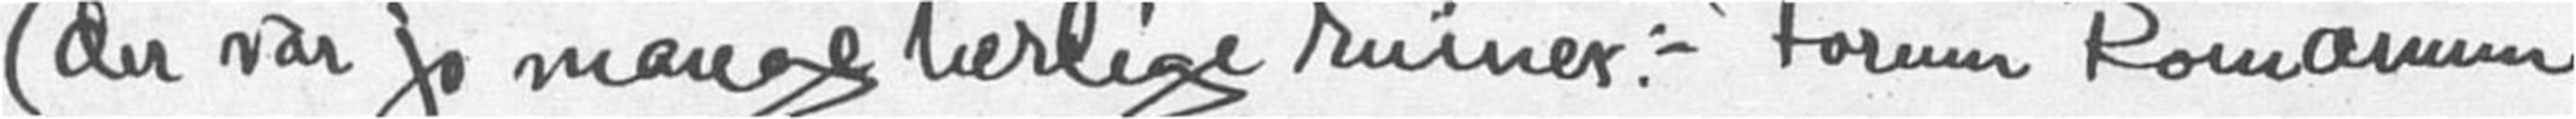(der var jo mange herlige ruiner : - Forum Romanum
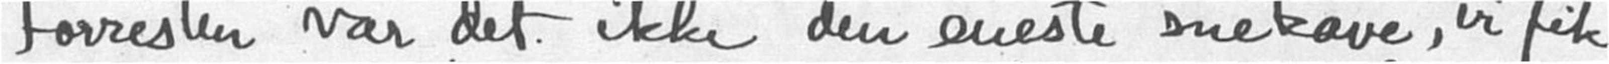Forresten var det ikke den eneste snekave, vi fik
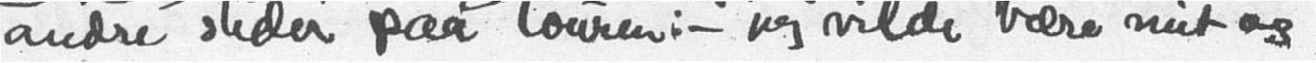andre steder paa touren : - jeg vilde bære mit og
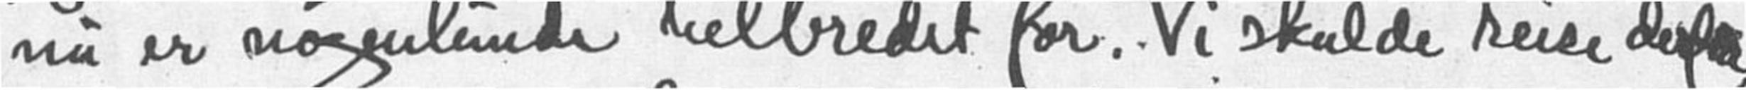nu er nogenlunde helbredet for. Vi skulde reise derfra,
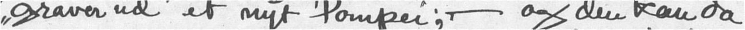"graver ud" et nyt Pompei; -og den kan da
